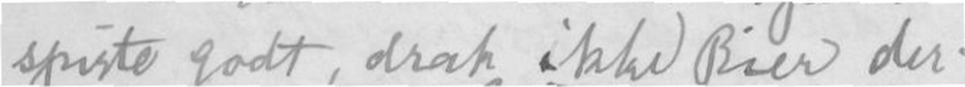spiste godt, drak ikke Bier der-
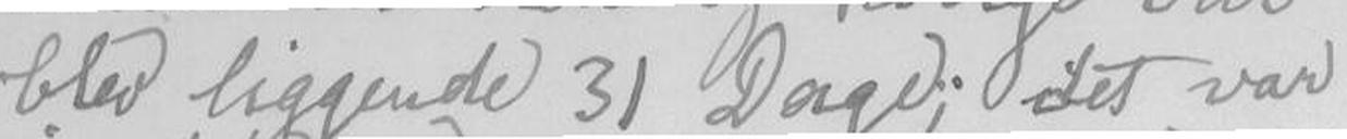blev liggende 31 Dage; det var
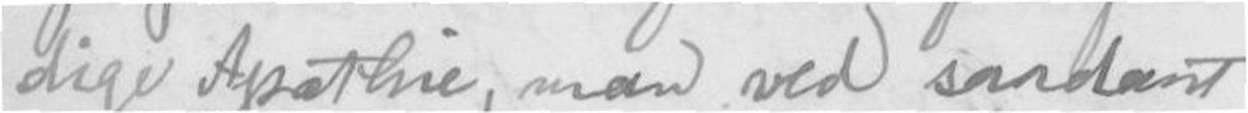dige Apathie, man ved saadant
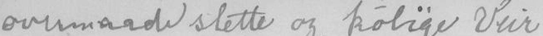overmaade slette og kølige Veir
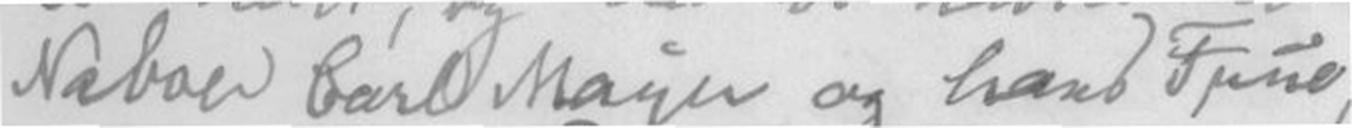Naboer Carl Mayer og hans Frue,
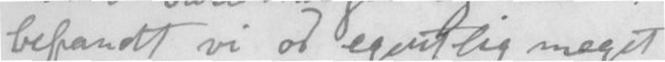befandt vi os egentlig meget
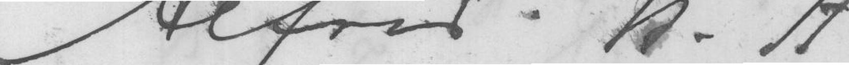A. Wilhelm Hansen
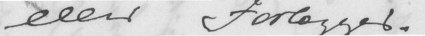eller Forlegger.
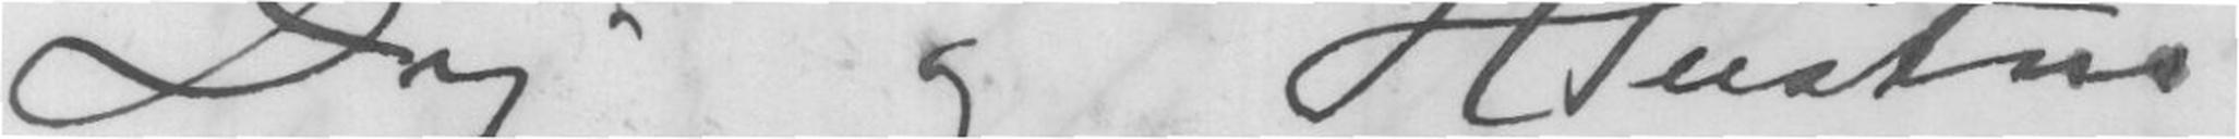Dig og Hustru
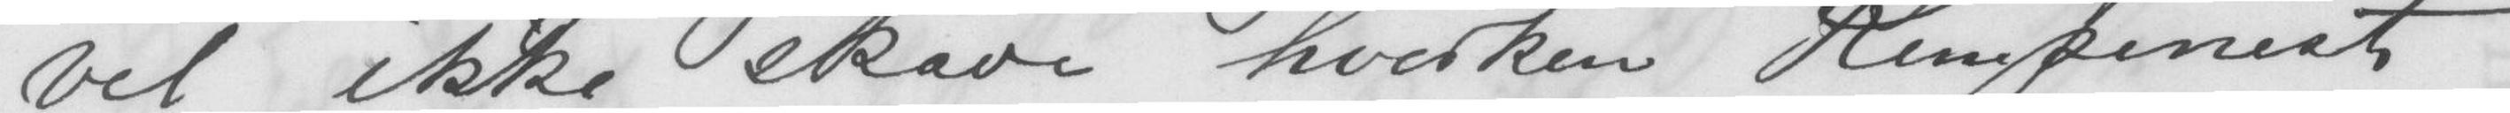vel ikke skade hverken Komponist
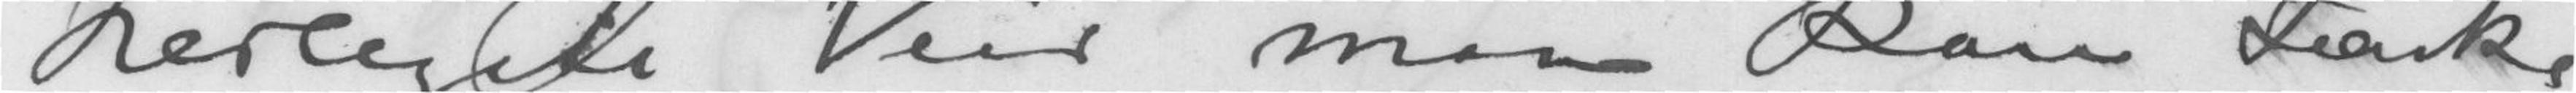herligete Veir man kan tænke
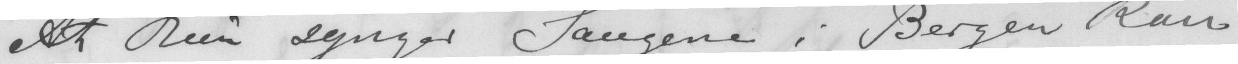At hun synger Sangene i Bergen kan
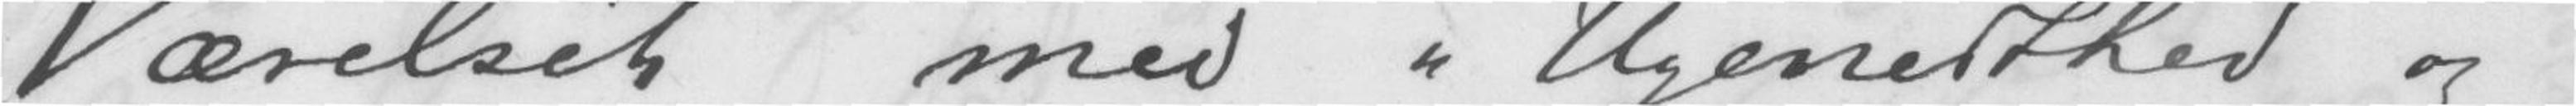Værelset med "Ugenedthed og
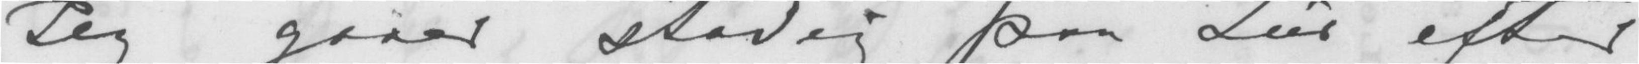Jeg gaar stadig paa Lur efter
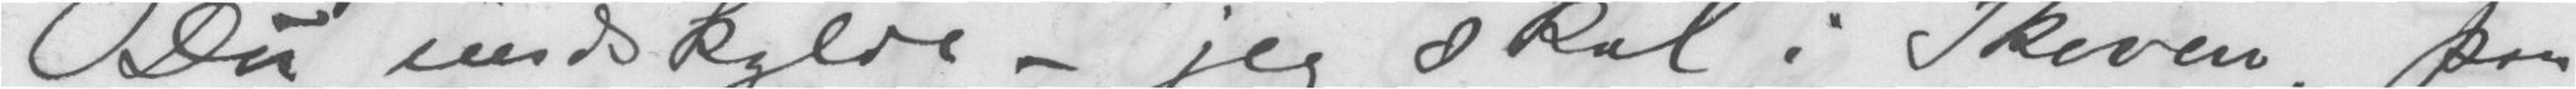Du undskylde - jeg skal i Skeven, paa
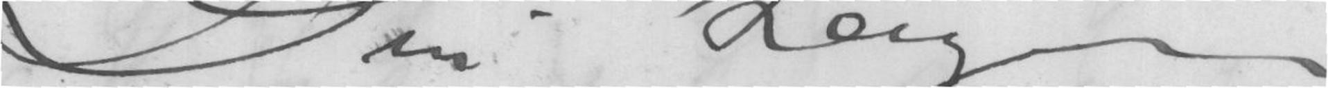Din hengivne
